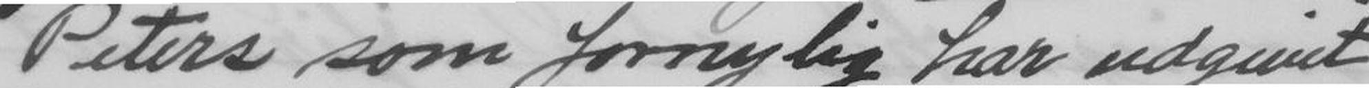Peters som fornylig har udgivet
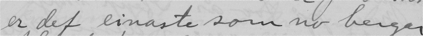er det einaste som no bergar
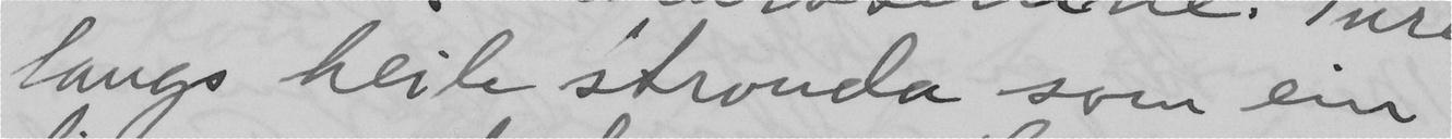langs heile stronda som ein
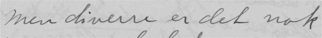Men diverre er det nok
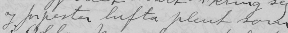og forpester lufta plent som
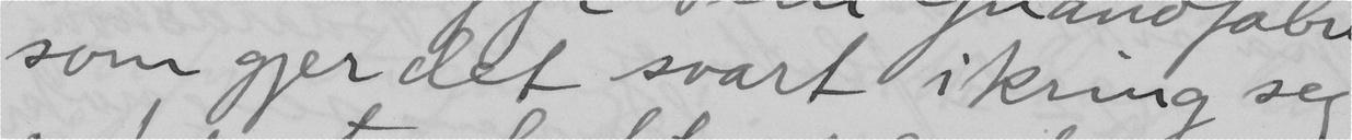som gjer det svart ikring seg
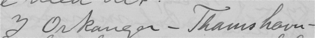I Orkanger – Thams havn –
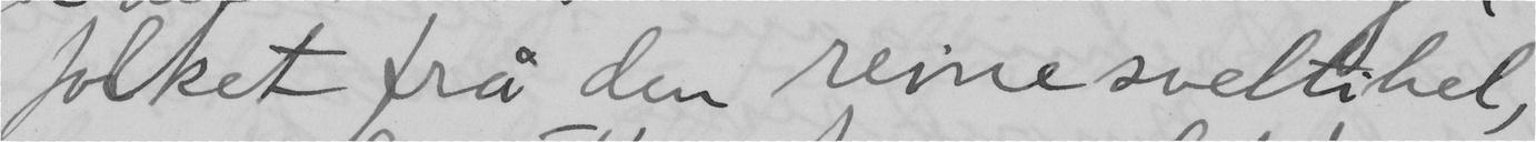folket frå den reine sveltihel,
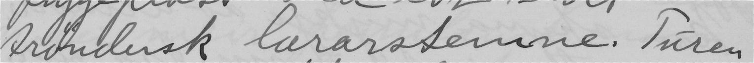tröndersk lærarstemne. Turen
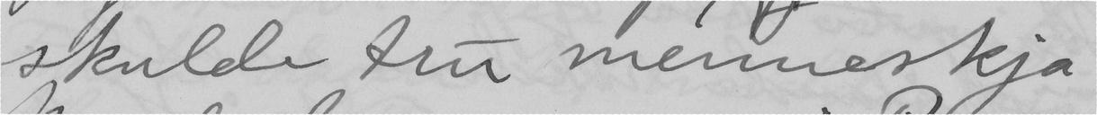skulde tru menneskja
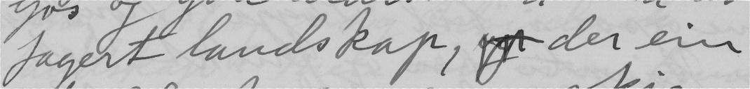fagert landskap,  der ein
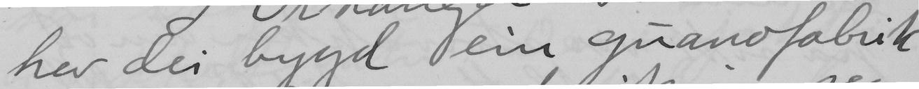hev dei bygd ein guanofabrik
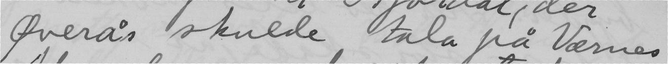Overås skulde tala på Værnes
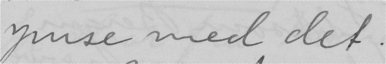ymse med det.
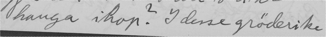hanga ihop? I desse gröderike
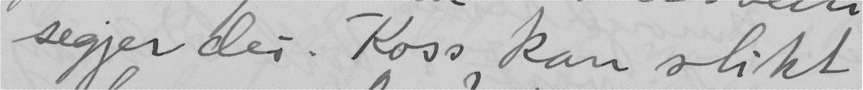segjer dei. Koss kan slikt
